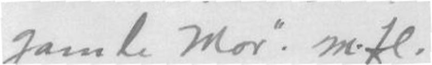Gamle Mor." m.fl.
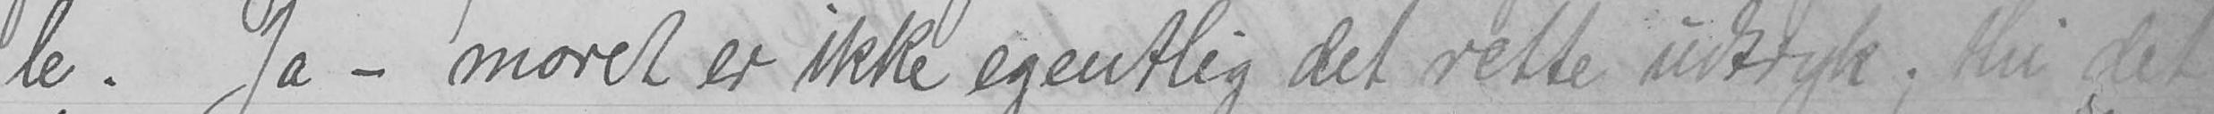le. Ja - moret er ikke egentlig det rette udtryk; thi det
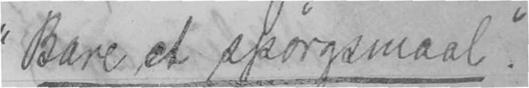"Bare et spørgsmaal."
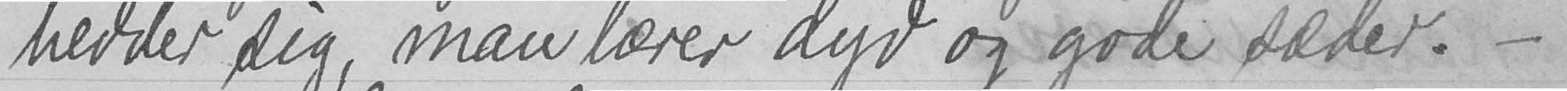hedder sig, man lærer dyd og gode sæder. -
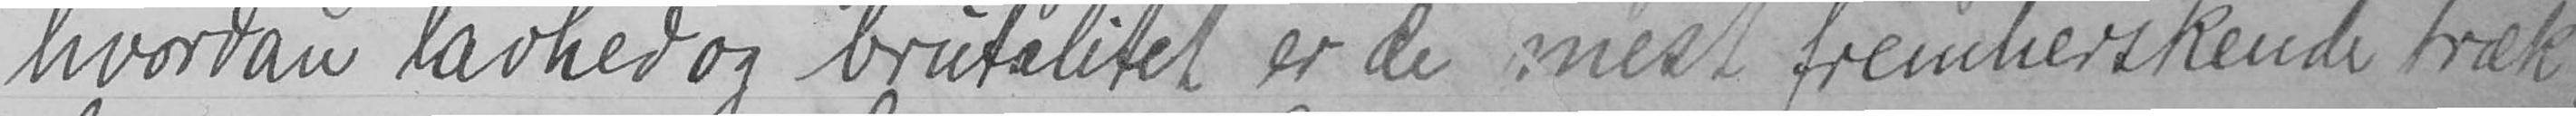hvordan lavhed og brutalitet er de mest fremherskende træk
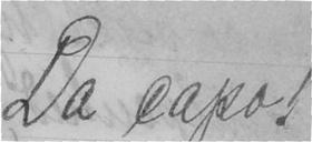Da capo!
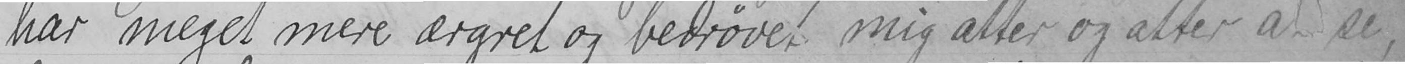har meget mere ærgret og bedrøvet mig atter og atter at se,
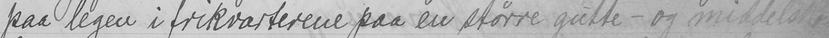paa legen i frikvarterene paa en større gutte- og middelsko-
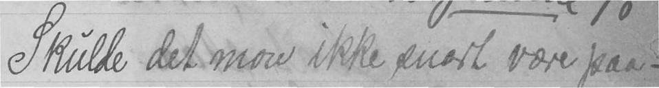Skulde det mon ikke snart være paa-
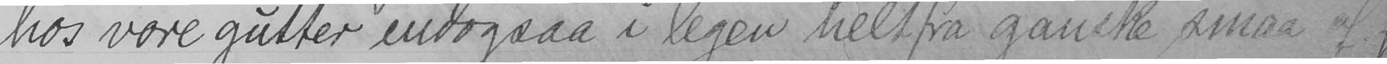hos vore gutter endogsaa i legen helt fra ganske smaa af
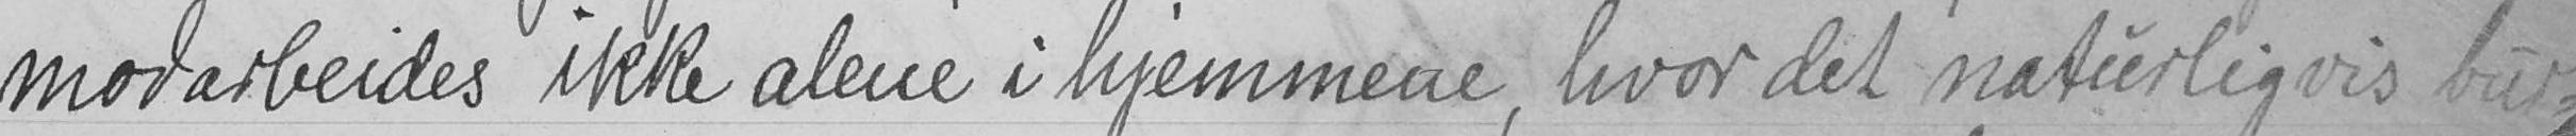modarbeides ikke alene i hjemmene, hvor det naturligvis bur-
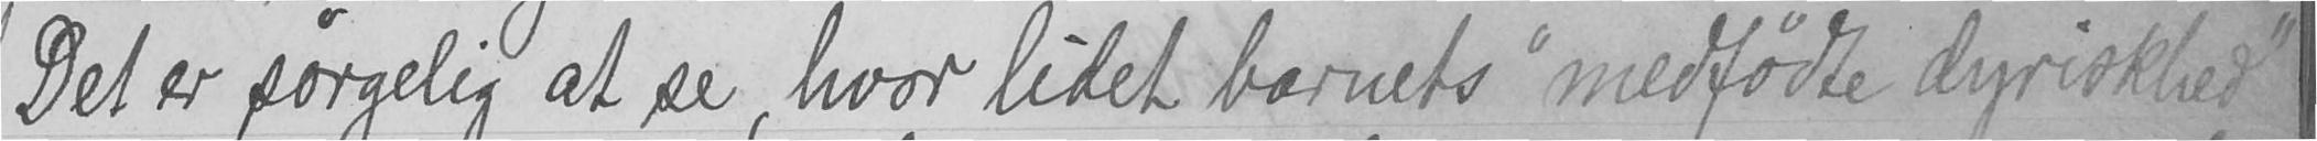Det er sørgelig at se, hvor lidet barnets "medfødte dyriskhed"
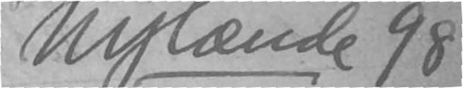Nylænde 98
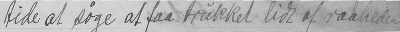tide at søge at faa trukket lidt af raaheden
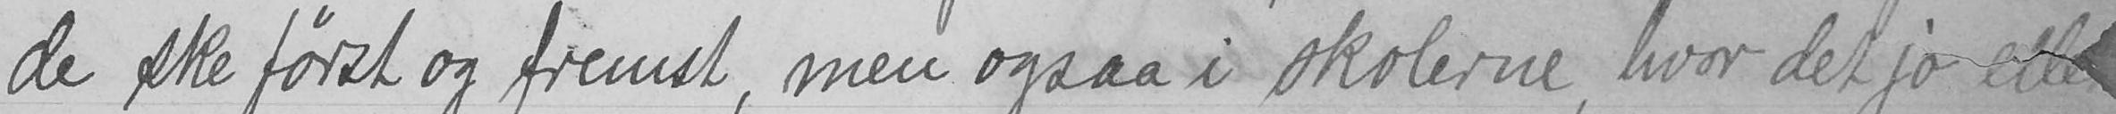de ske først og fremst, men ogsaa i skolerne, hvor det jo ellers
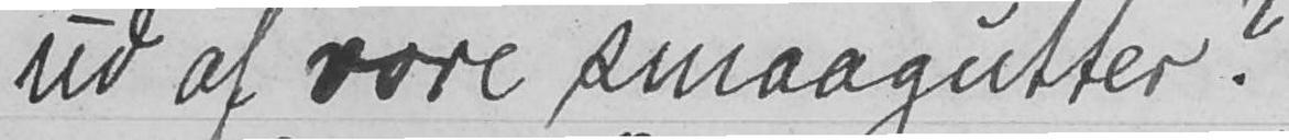ud af vore smaagutter?
